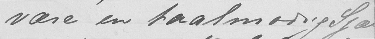være en taalmodig Sjæl
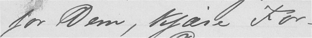for Dem, Kjære For-
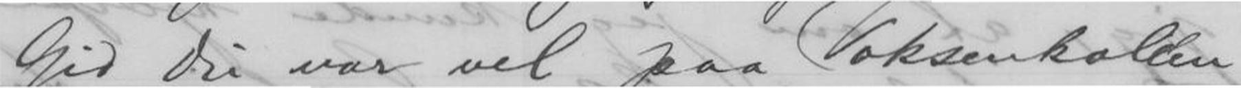Gid du var vel paa Voksenkollen
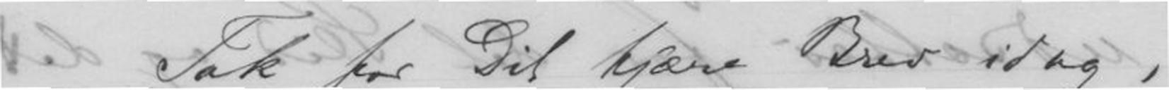Tak for Dit kjære Brev idag,
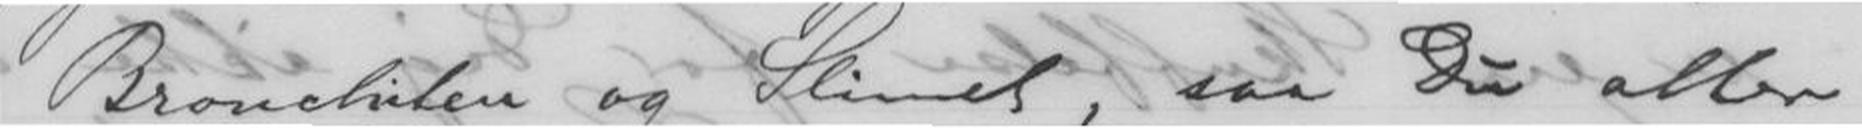Bronchiten og Slimet, saa Du atter
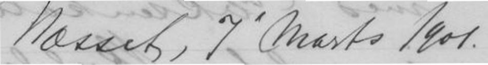Næsset 7 Marts 1901.
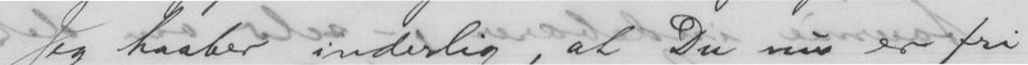Jeg haaber inderlig, at Du nu er fri
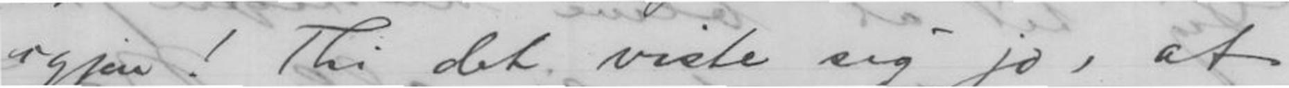igjen! Thi det viste sig jo, at
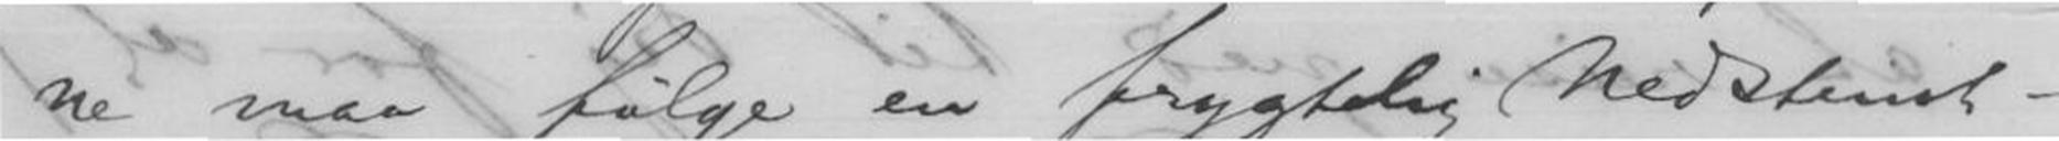ne maa følge en frygtelig Nedstemt-
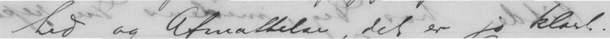hed og Afmattelse, det er jo klart.
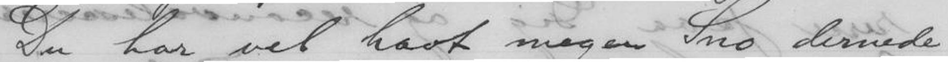Du har vel havt megen Sno dernede
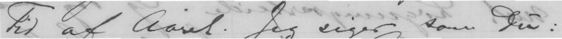Tid af Aaret. Jeg siger som Du:
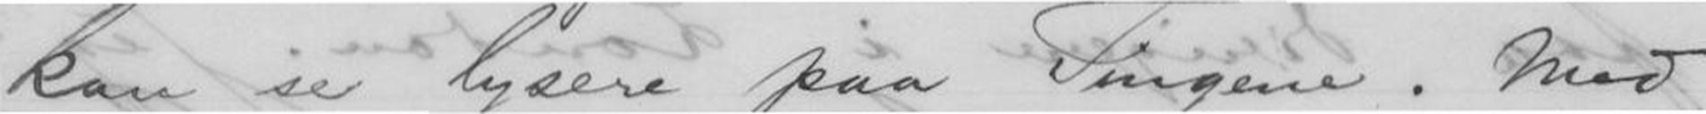kan se lysere paa Tingene. Med
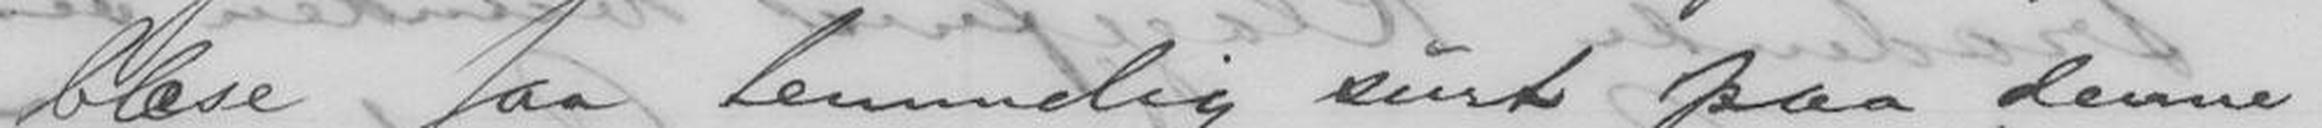blæse saa temmelig surt paa denne
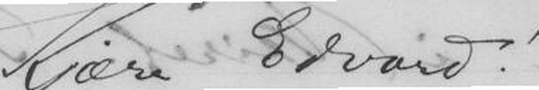Kjære Edvard!
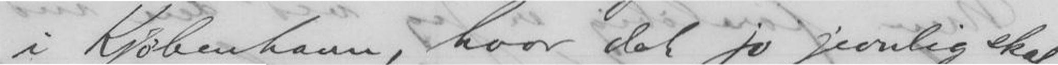i Kjøbenhavn, hvor det jo jevnlig skal
